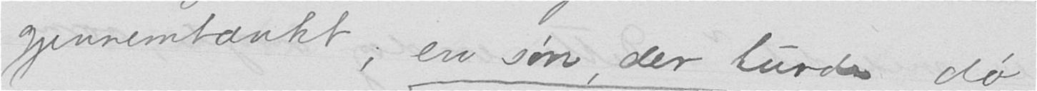gjennemtænkt, en søn, der turde dø
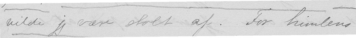vilde jeg være stolt af. For himlens
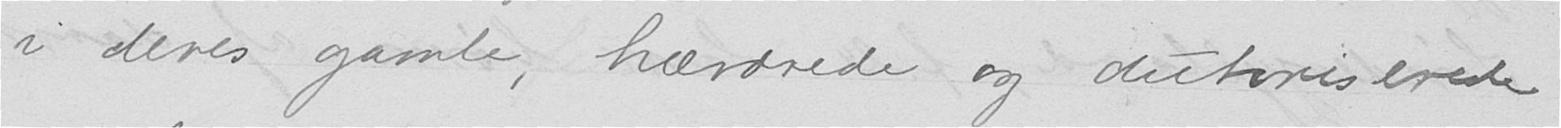i deres gamle, hævdede og autoriserede
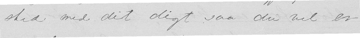sted med dit digt saa du vel er
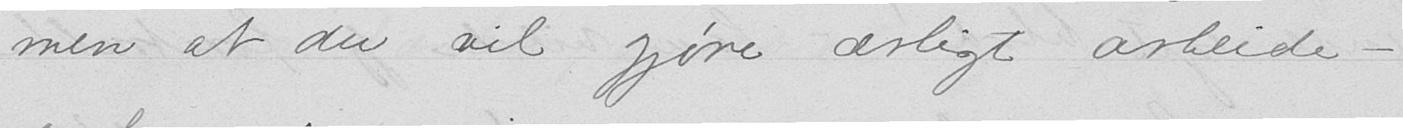men at du vil gjøre ærligt arbeide -
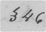§ 46
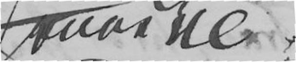Jonas Lie.
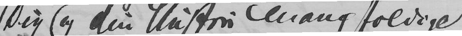Dig og din Hustru mangfoldige
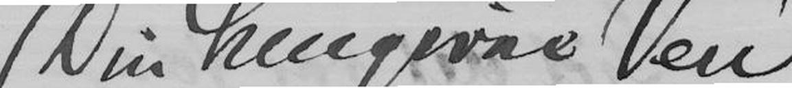Din hengivne Ven
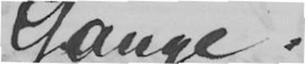Gange.
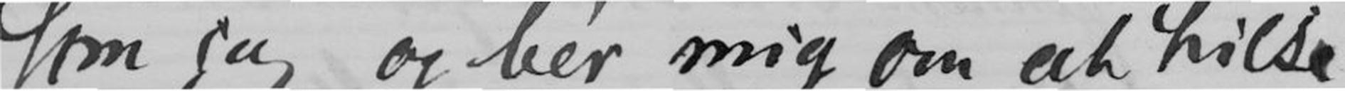Som jeg og ber mig om at hilse
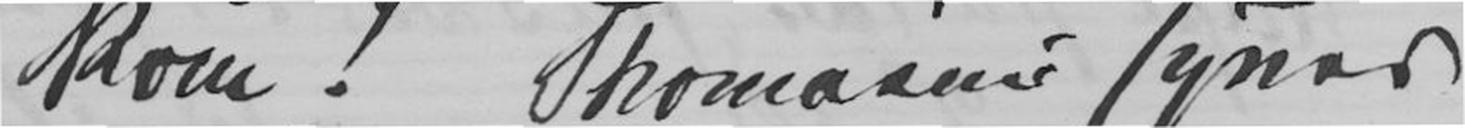som! Thomasine synes
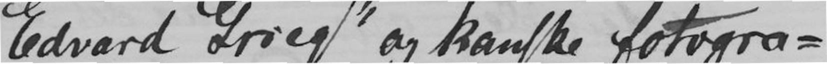Edvard Grieg" og kanske fotogra-
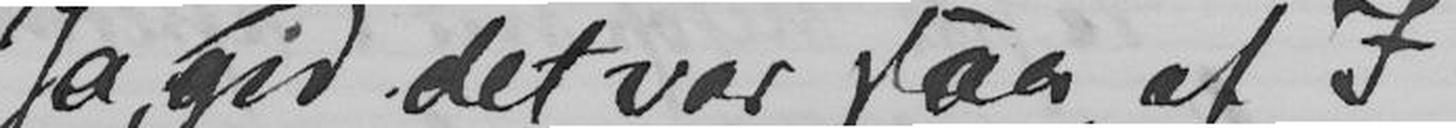Ja, gid det var saa, at I
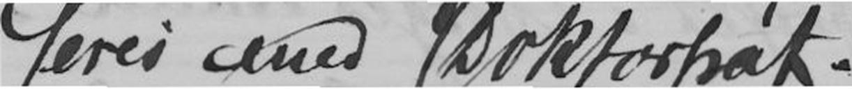feres med Doktorhat.
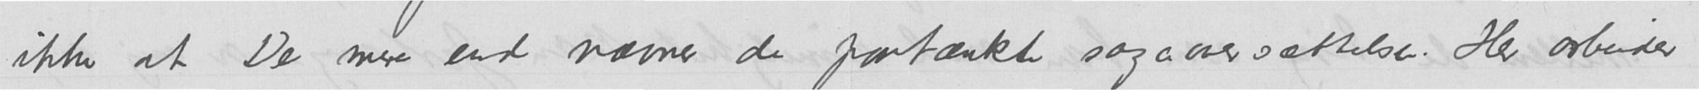ikke at De mere end nævner de paatænkte sagaoversættelser. Her arbeider
